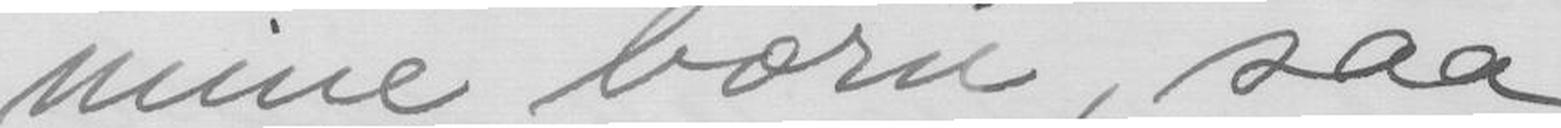mine børn, saa
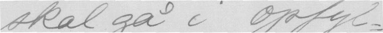skal gå i opfyl-
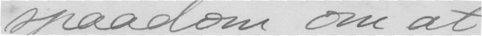spaadom om at
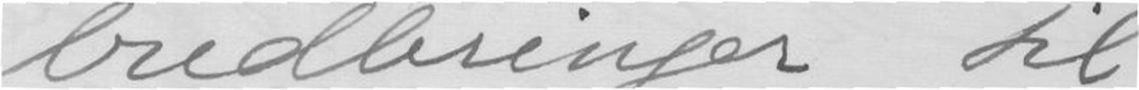budbringer til
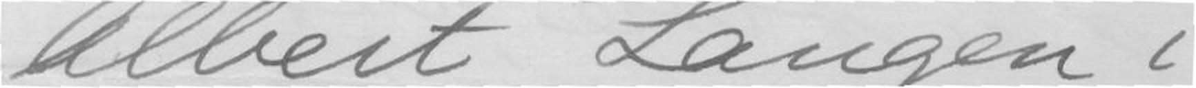Albert Langen i
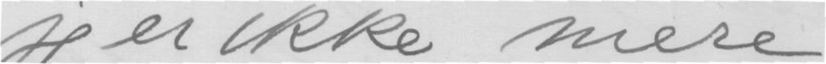jeg er ikke mere
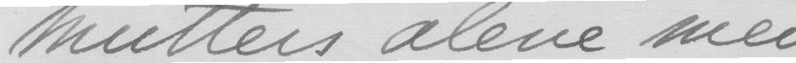mutters alene med
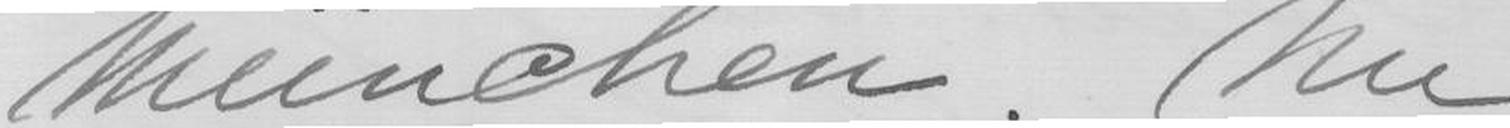München. Nu
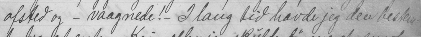afsted og - vaagnede! - I lang tid havde jeg den bestem-
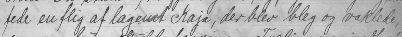fede en flig af lagenet Kaja, der blev bleg og vaklede,
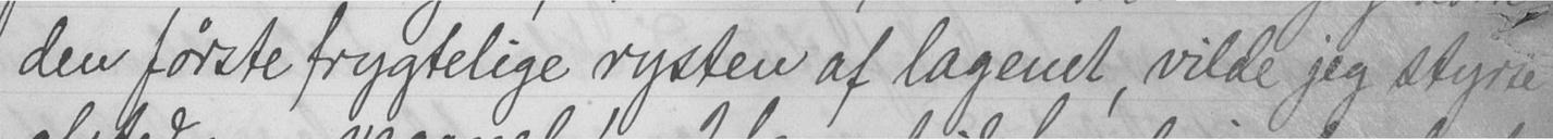den første frygtelige rysten af lagenet, vilde jeg styrte
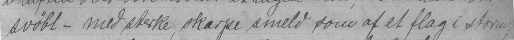svøbt - med sterke, skarpe smeld som af et flag i storm;
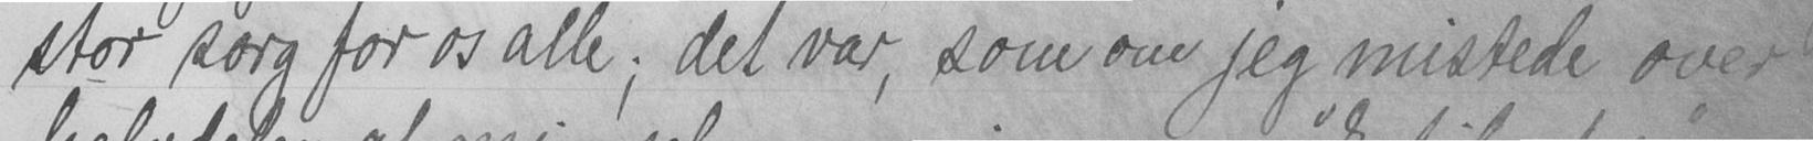stor sorg for os alle; det var, som om jeg mistede over
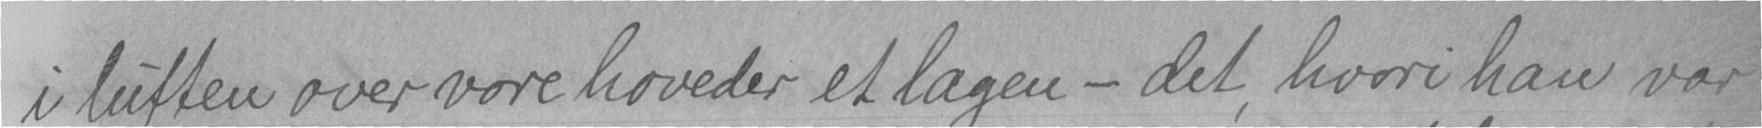i luften over vore hoveder et lagen - det, hvori han var
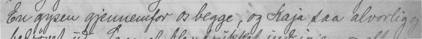En gysen gjennemfor os begge, og Kaja saa alvorlig og
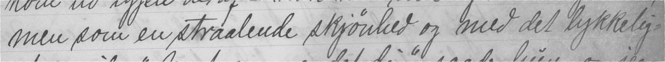men som en straalende skjønhed og med det lykkelig-
